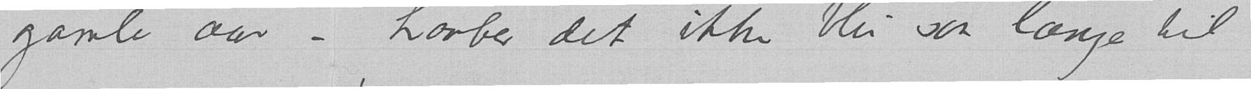gamle aar – haaber det ikke blir saa længe til
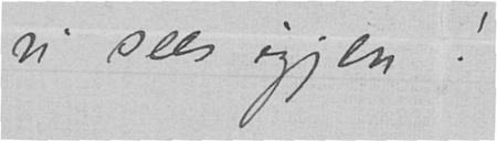vi sees igjen!
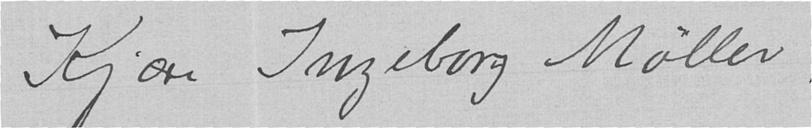Kjære Ingeborg Møller,
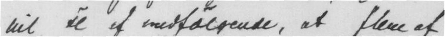vil se af medfølgende, at flere af
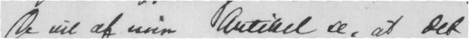De vil af min Artikel se, at det
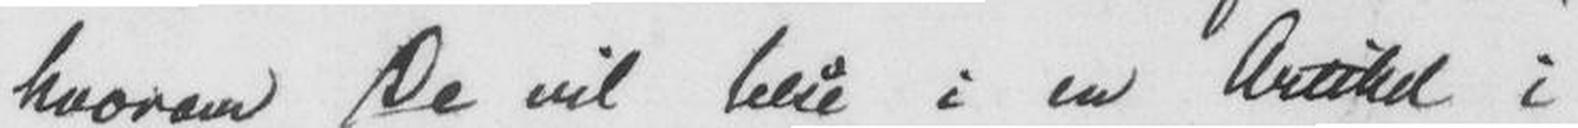hvorover De vil lese i en Arttikel i
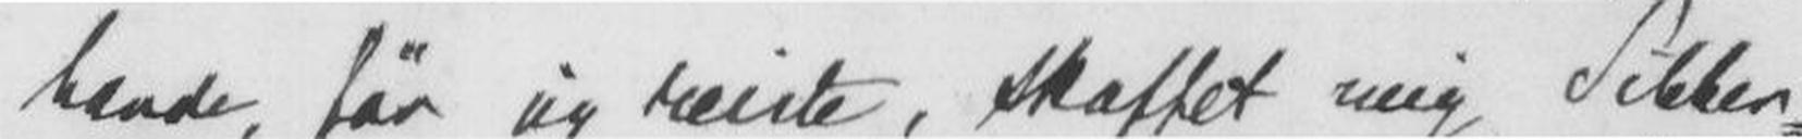havde, fär jeg reiste; skaffet mig Sikker-
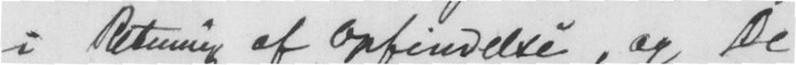i Retning af opfindelse, og De
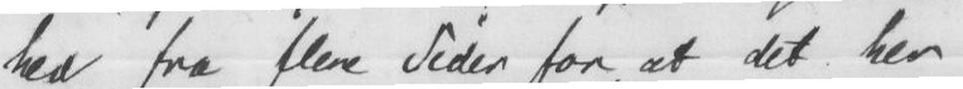hed fra flere Sider for, at det her
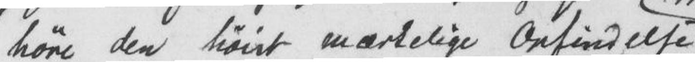høre den høist mærkelige
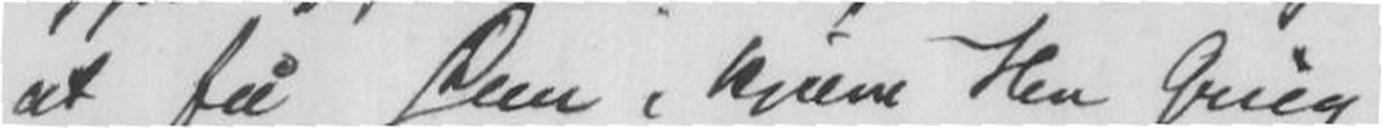at få Dem, kjære Herr Grieg
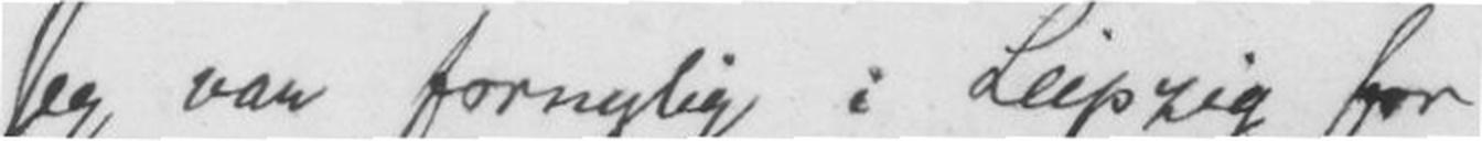Jeg var fornylig i Leipzig for
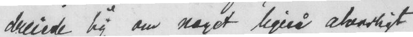dreiede sig om noget ligeså alvorligt
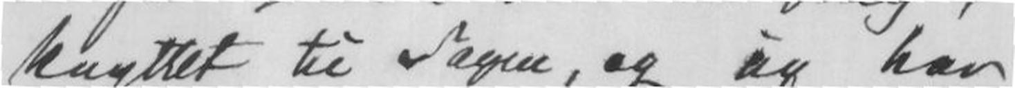knyttet til Sagen, og jeg har
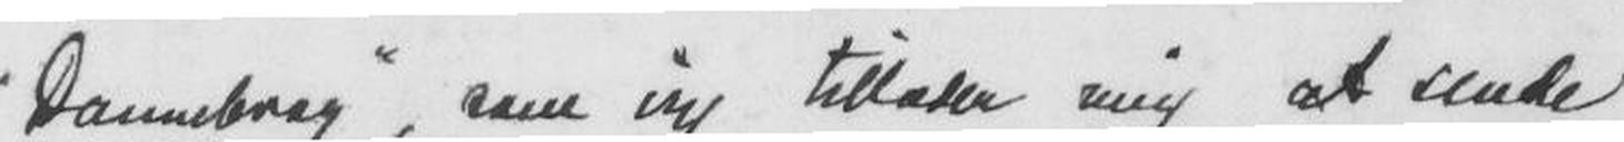Damsbrag, som jeg tillader mig at sende
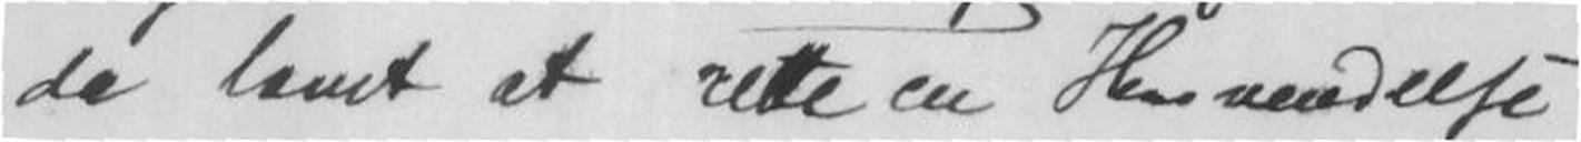da lovet at rette en Henvendelse
